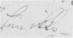hun ikke -
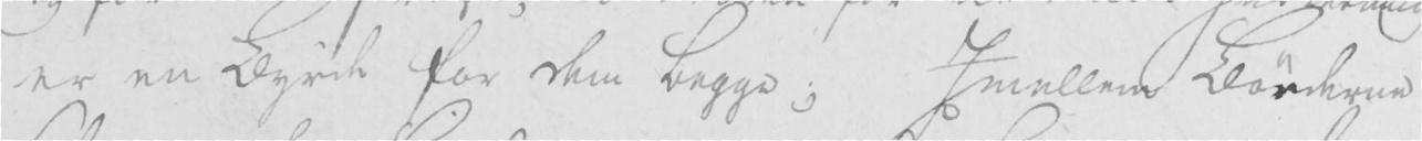er en Byrde for dem begge; Imellem Bønderne
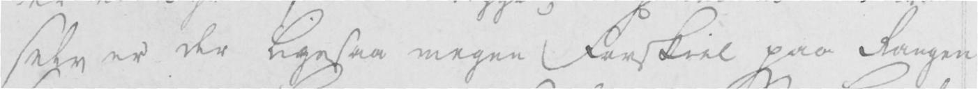selv er der ligesaa megen forskiel paa Rangen
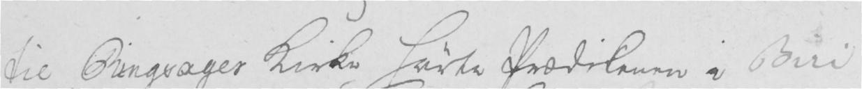til Ringsager Kirke hørte Prædikenen i Biri
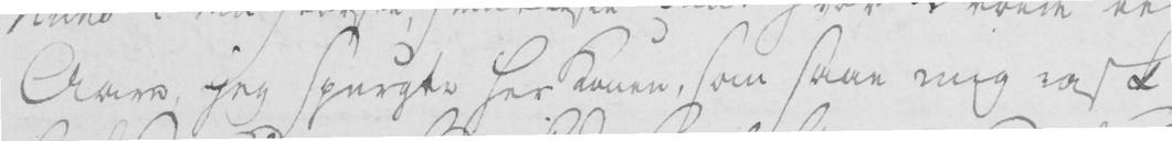Aare, jeg spurgte her Konen, som saae mig rask,
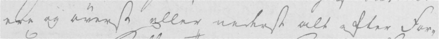ere og øverst eller nederst alt efter For-
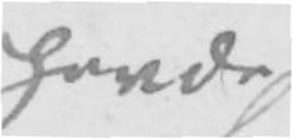havde
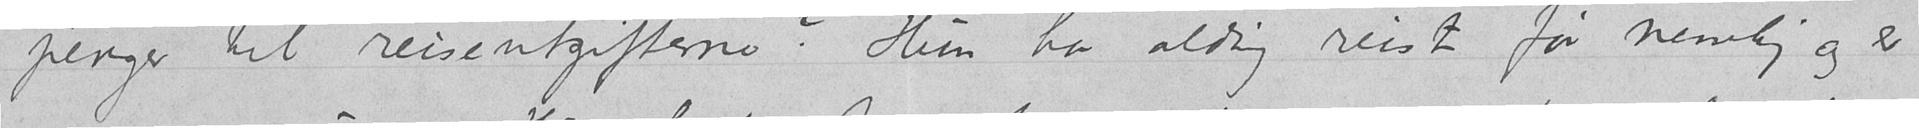penger til reiseutgifterne? Hun har aldrig reist før nemlig og er
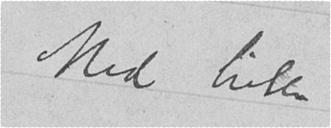Med hilsen
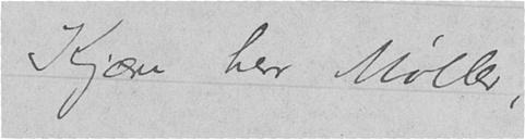Kjære her Møller,
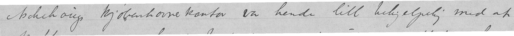Aschehougs kjøbenhavnerkontor var hende litt behjelpelig med at
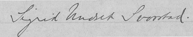Sigrid Undset Svarstad
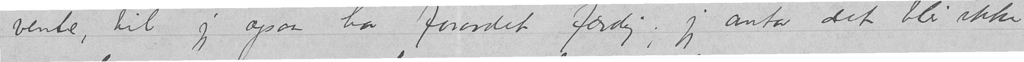vente, til jeg ogsaa har forordet færdig; jeg antar det blir ikke
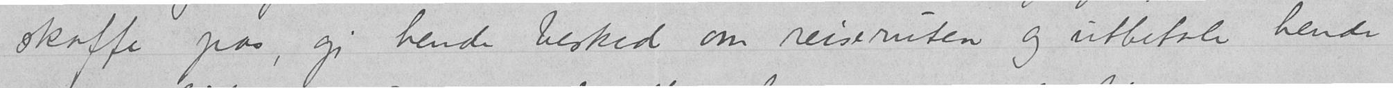skaffe pas, gi hende besked om reiseruten og utbetale hende
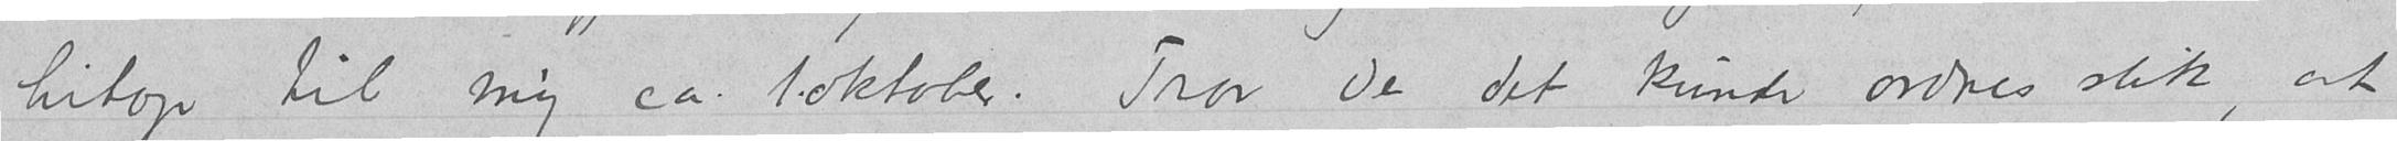hitop til mig ca. 1. oktober. Tror De det kunde ordnes slik, at
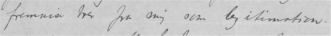fremvise brev fra mig som legitimation.
